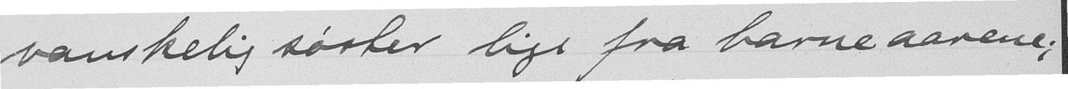vanskelig søster lige fra barneaarene;
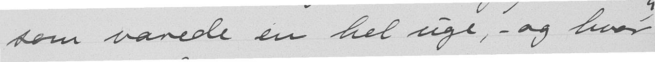som varede en hel uge, - og hvor
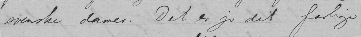svenske damer. Det er jo det farlige
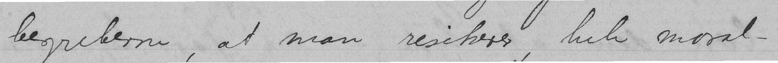begreberne, at man resikerer, hele moral-
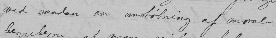med saadan en omstøbning af moral-
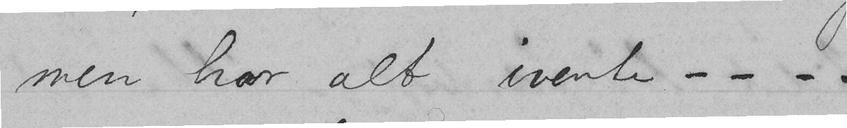men har alt ivente — — — —
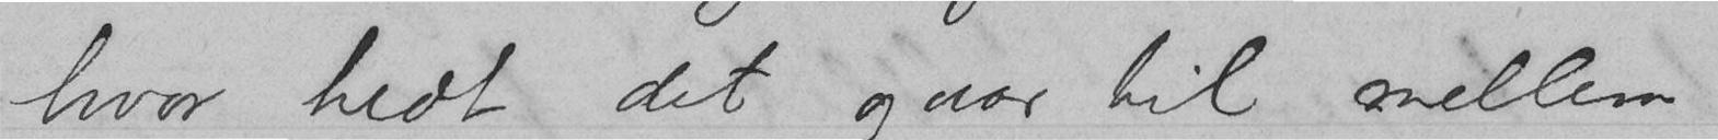hvor hedt det gaar til mellem
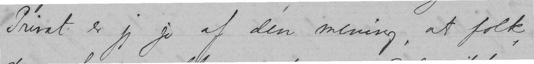Privat er jeg jo af den mening, at folk,
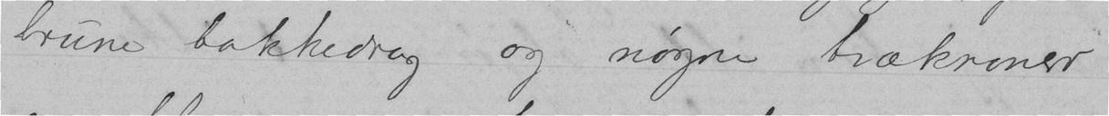brune bakkedrag og nøgne trækroner
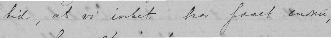tid, at vi intet har faaet endnu,
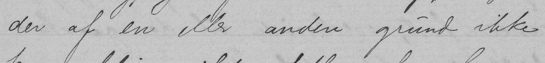der af en eller anden grund ikke
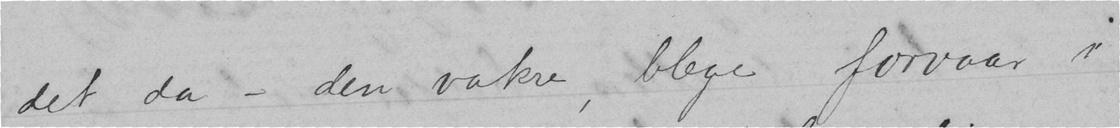det da — den vakre, blege forvaar i
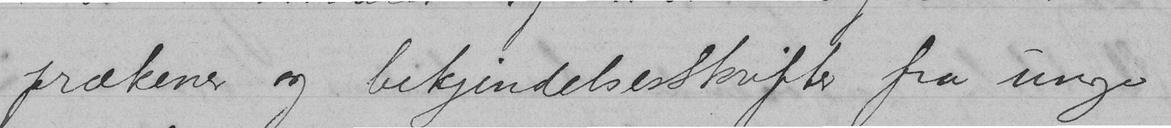prækener og bekjendelsesskrifter fra unge
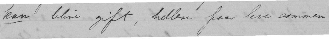kan blive gift, heller faar leve sammen
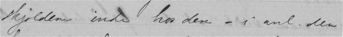skjoldene inde hos dere — i anl. den
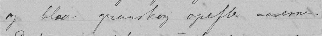og blaa granskog opefter aaserne.
